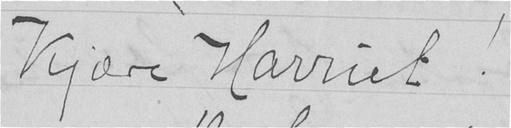Kjære Harriet!
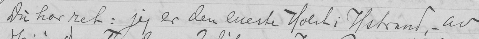Du har ret: jeg er den eneste Holst i Hstrand, - av
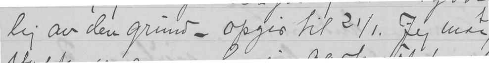lig av den grund - opgir til 21/1. Jeg maa
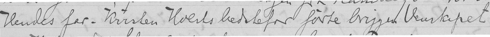Hendes far - Kristen Holsts bedstefar førte bryggen Venskapet
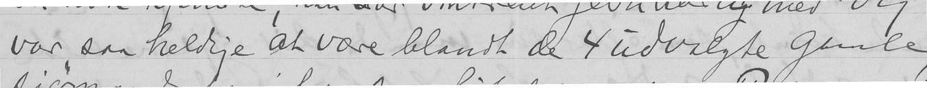var saa heldige at være blandt de 4 udvalgte gamle
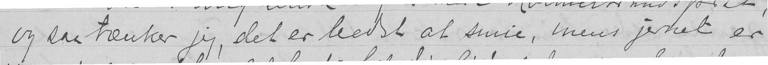og saa tænker jeg, det er bedst at smie, mens jernet er
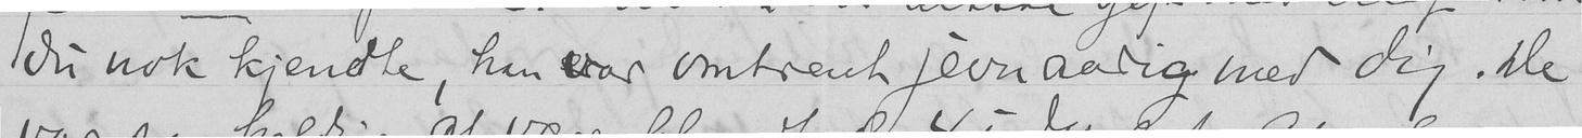du nok kjendte, han var omtrent jevnaarig med dig. De
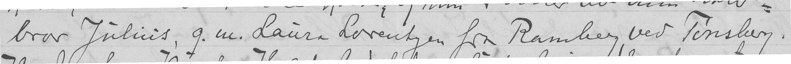bror Julius, g. m. Laura Lorentzen fra Ramberg ved Tønsberg.
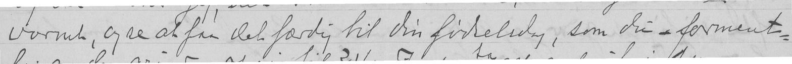varmt, og se at faa det færdig til din fødselsdag, som du - forment-
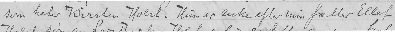som heter Kirsten Holst. Hun er enke efter min fætter Ellef
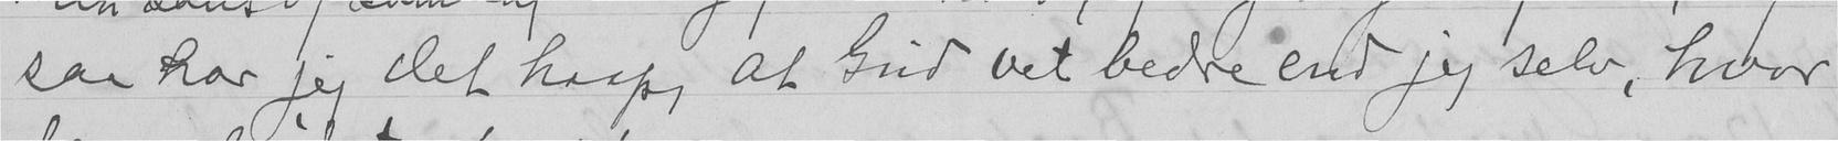saa har jeg det haap, at Gud vet bedre end jeg selv, hvor
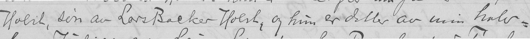Holst, søn av Lars Backer Holst, og hun er datter av min halv-
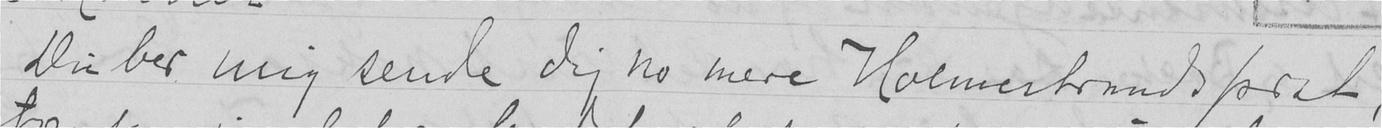Du ber mig sende dig no mere Holmestrands prat,
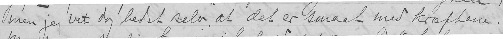men jeg vet dog bedst selv, at det er smaat med kræftene.
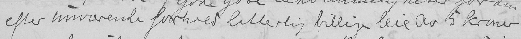efter nuværende forhold latterlig billige leie av 5 kroner
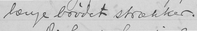længe brødet strækker.
